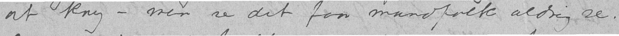at kny - men se det faar mandfolk aldrig se.
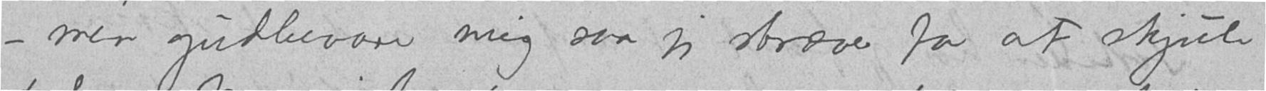- men gudbevare mig saa jeg stræver for at skjule
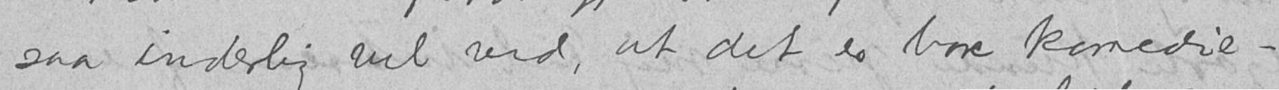saa inderlig vel ved, at det er bare komedie -
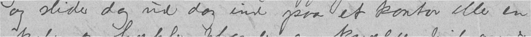og slider dag ud dag ind paa et kontor eller en
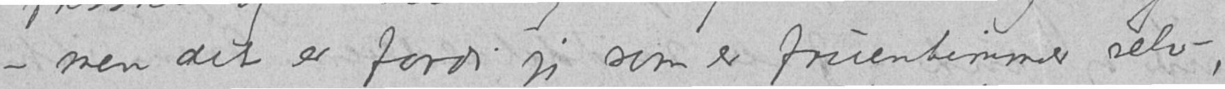- men det er fordi jeg som er fruentimmer selv -,
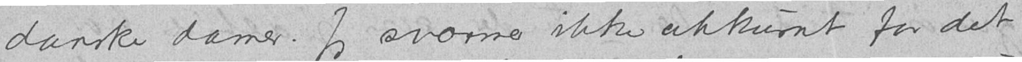danske damer. Jeg sværmer ikke akkurat for det
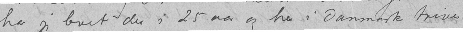har jeg levet der i 25 aar og her i Danmark trives
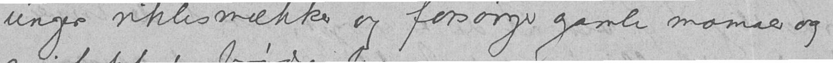ungers siklesmækker og forsørger gamle mamaer og
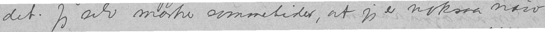det. Jeg selv mærker sommetider, at jeg er noksaa naiv
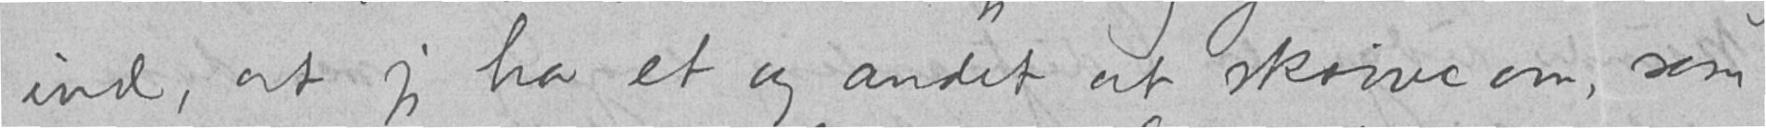ind, at jeg har et og andet at skrive om, som
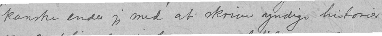kanske ender jeg med at skrive yndige historier
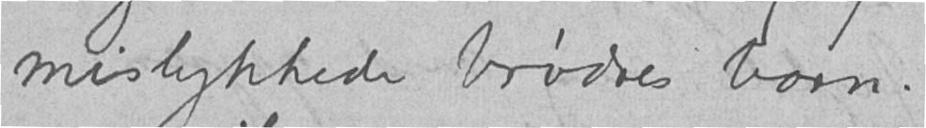mislykkede brødres barn.
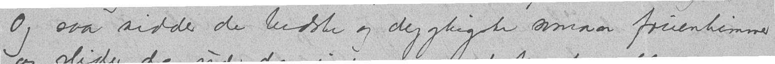Og saa sidder de bedste og dygtigste smaa fruentimmer
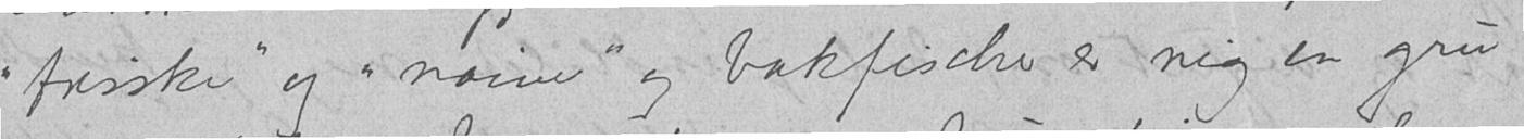"frisske og "naive" og bakfischer er mig en gru
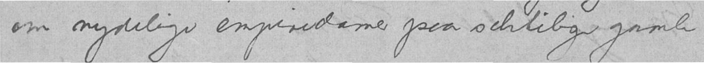om nydelige empiredamer paa schtilige gamle
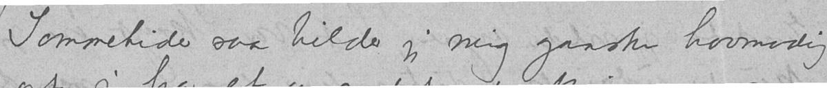Sommetider saa bilder jeg mig ganske hovmodig
